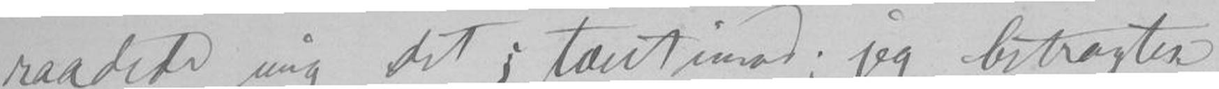raadede mig det; tvertimod; jeg betragter
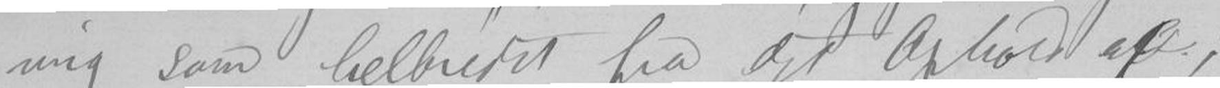mig som helbredet fra det Ophold af;
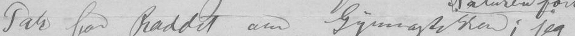Tak for Raadet om Gymnastikken; jeg
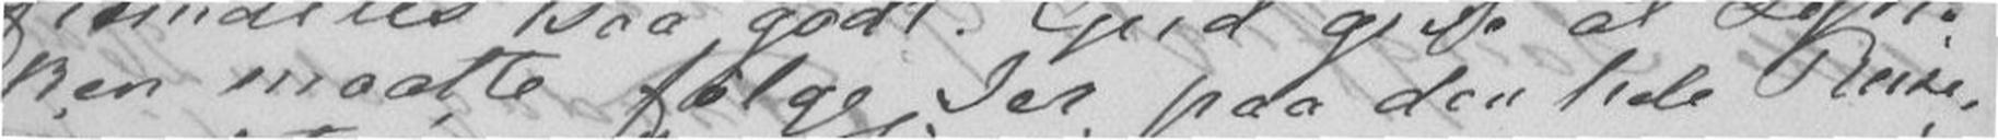ken maatte følge Jer paa den hele Reise,
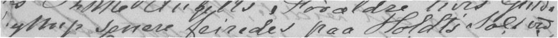ryllup senere feiredes paa Holdt's Sal ved
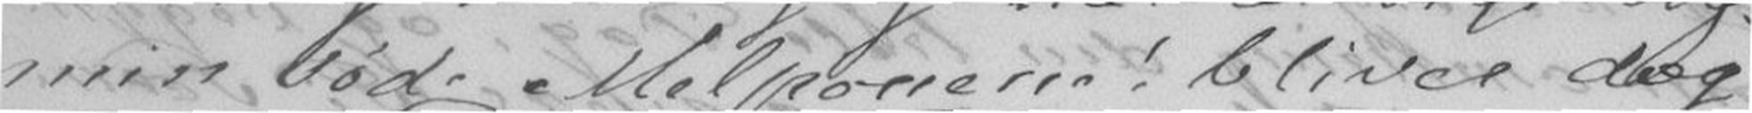min søde Melponene! bliver dog
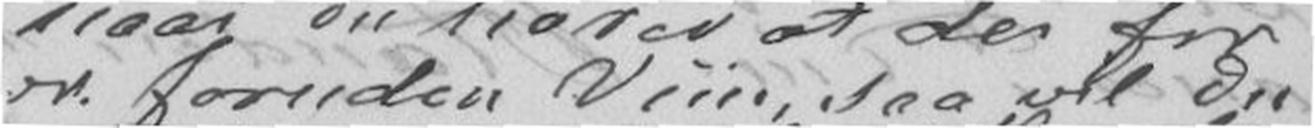or foruden Viin, saa vil Du
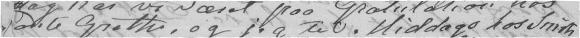Tante Grethe, og jeg til Middags hos Smits,
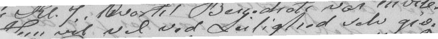Hun vil ved Leilighed selv give
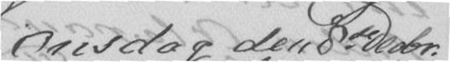Onsdag de 8de Decbr.
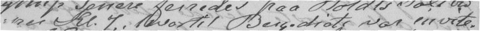er Kl.7., hvortil Benedicte var invite-
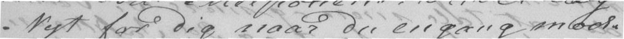Nyt for Dig naar Du engang mod-
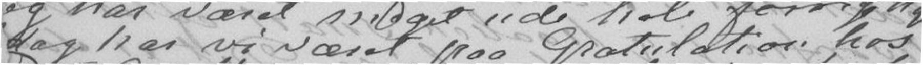Idag har vi været paa Gratulation hos
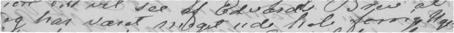jeg har været meget ude hele forrige Uge.
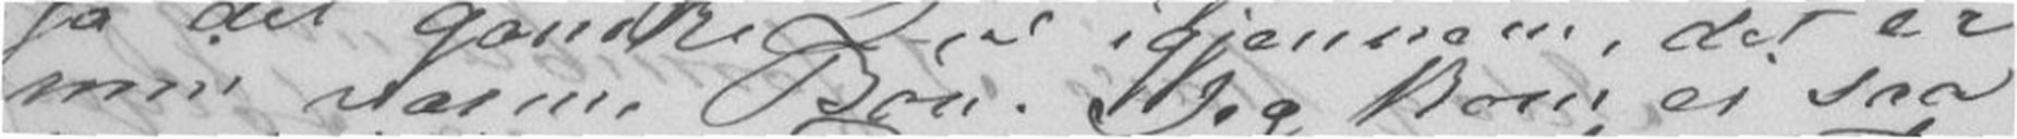min varme Bøn. Jeg kom ei saa
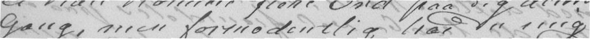gang, men formodentlig har Du mig
